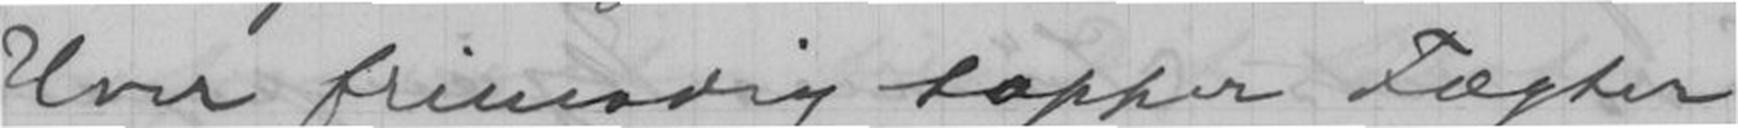"Hver frimodig tapper Fægter
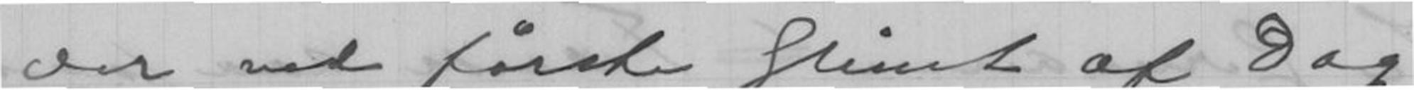der ved første Glimt af Dag
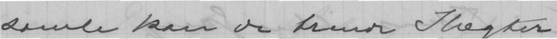samle kan de trende Slægter
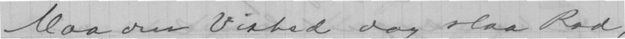Maa den Vished dog slaa Rod,
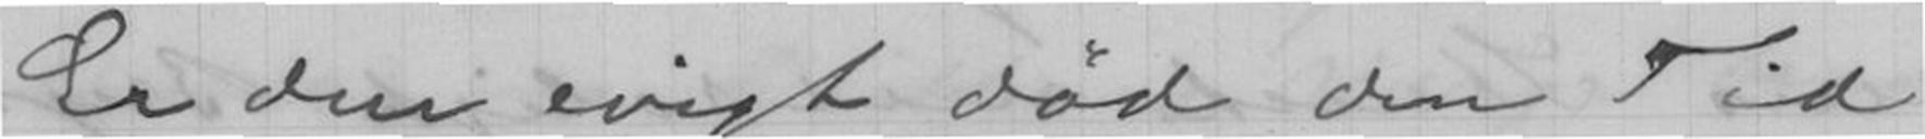Er den evigt Død den Tid,
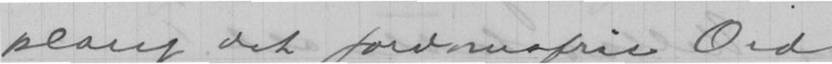klang det fordomsfrie Ord:
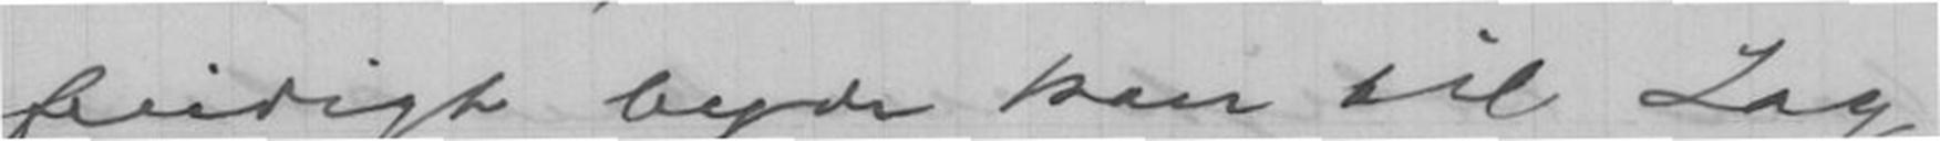freidigt byde kan til Lag
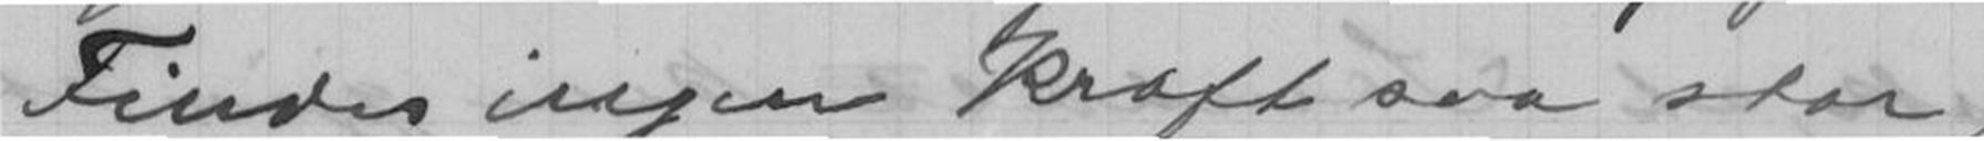Findes ingen Kraft saa stor,
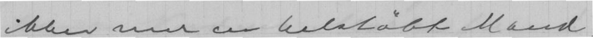ikke mer en helstøbt Mand,
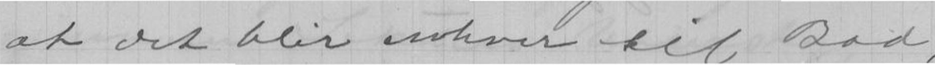at det blir enhver til Bod,
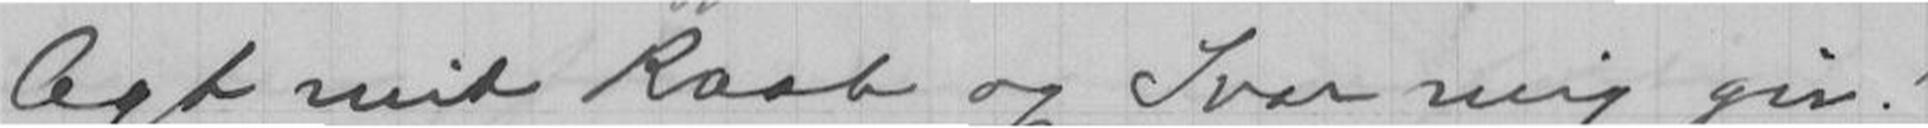Agt mit Raab og Svar mig giv!
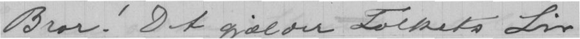Bror! Det gjælder Folkets Liv!
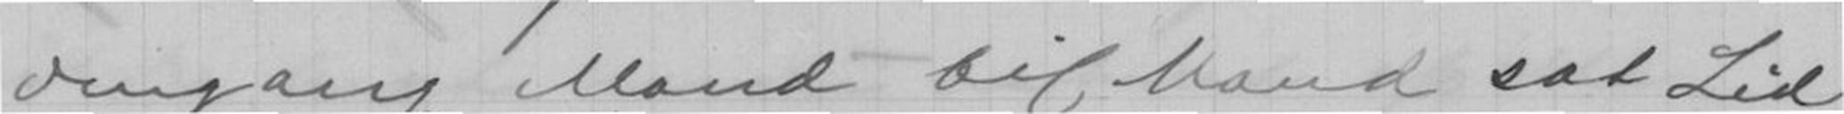dengang Mand tilhand sat Lid
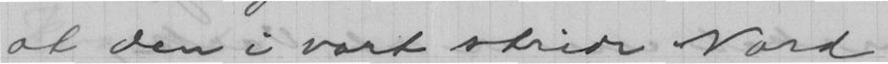at den i vort stride Nord
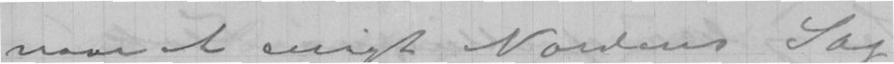naar et evigt Nordens Sag
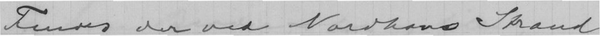Findes der ved Nordhavs Strand
